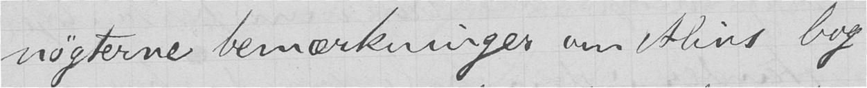nøgterne bemærkninger om Alins bog
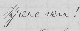Kjære ven!
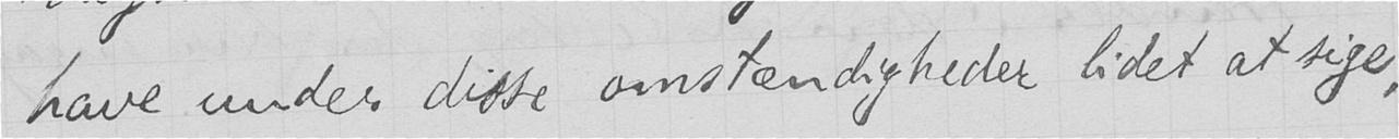have under disse omstændigheder lidet at sige,
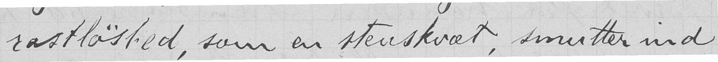rastløshed, som en stenskvæt, smutter ind
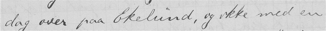dag over paa Ekelund, og ikke med en
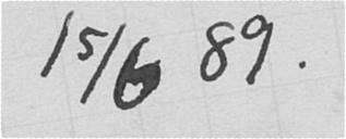15/6 89.
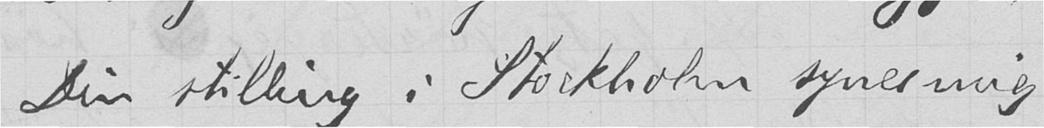Din stilling i Stockholm synes mig
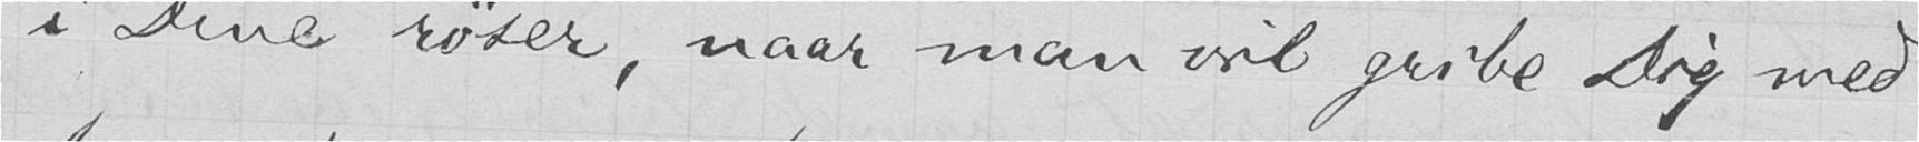i Dine røser, naar man vil gribe Dig med
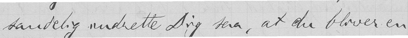sandelig indrette Dig saa, at du bliver en
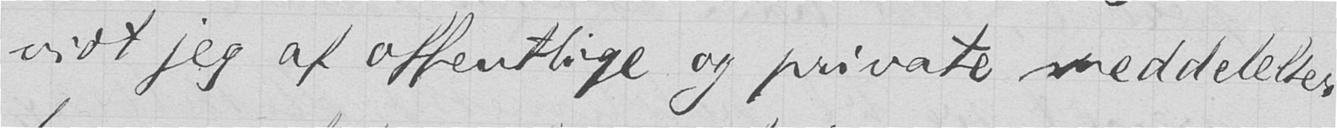vidt jeg af offentlige og private medddelser
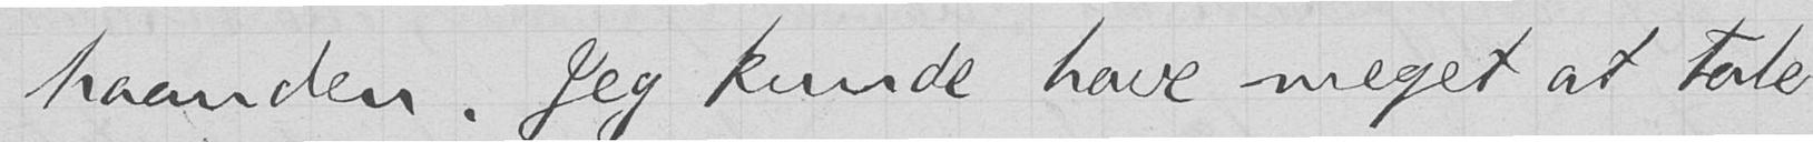haanden. Jeg kunde have meget at tale
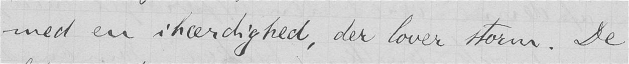med en ihærdighed, der lover storm. De
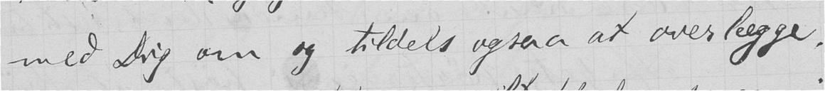med Dig om og tildels ogsaa at overlægge.
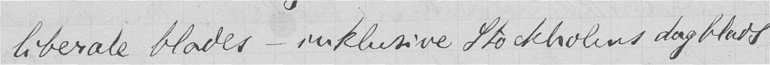liberale blades – inklusive Stockholms dagblads –
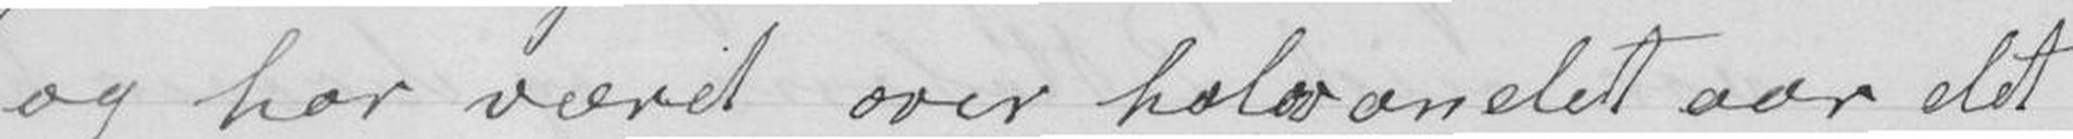og har været over halvandet aar det
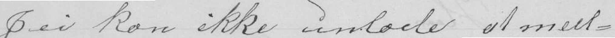Jei kan ikke unlade at med-
